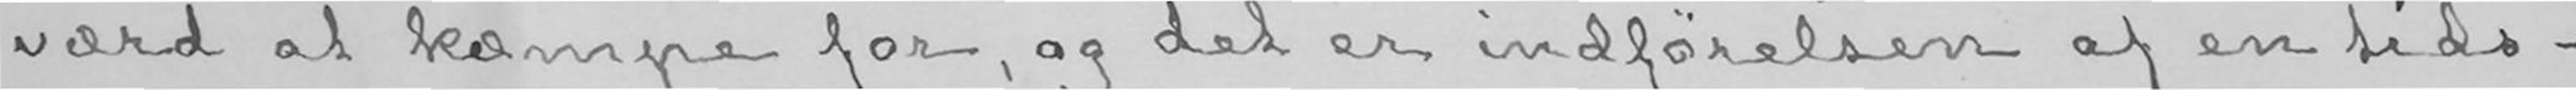værd at kæmpe for, og det er indførelsen af en tids-
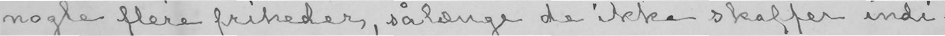nogle flere friheder, sålænge de ikke skaffer indi-
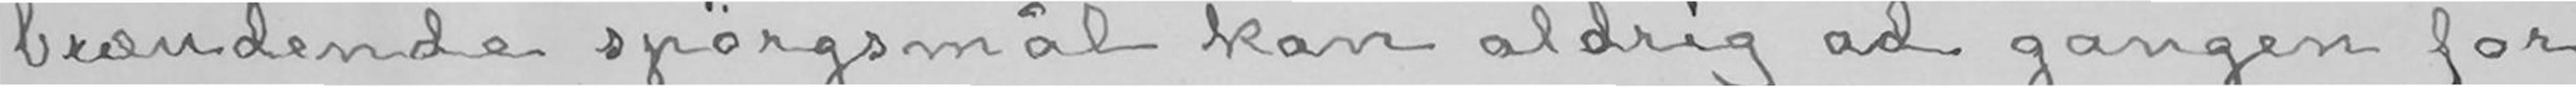brændende spørgsmål kan aldrig ad gangen for
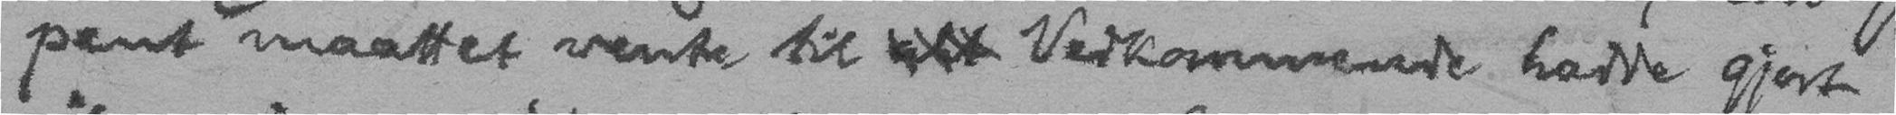pent maattet vente til alt Vedkommende hadde gjort
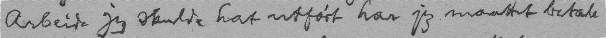Arbeide jeg skulde hat utført har jeg maattet betale
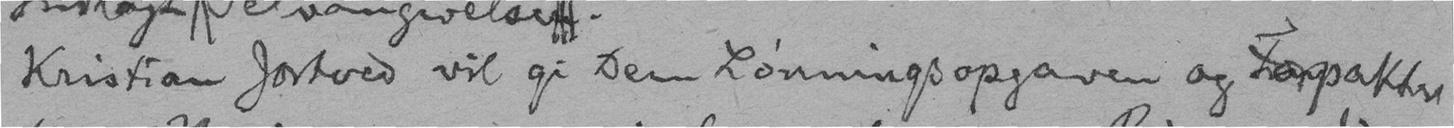Kristian Jortved vil gi Dem Lønningsopgaven og Forpakter
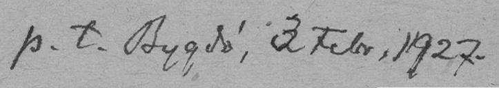p. t. Bygdø, 3 Febr. 1927.
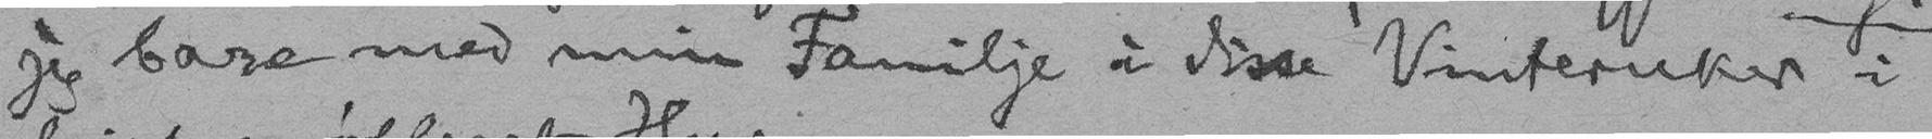jeg bare med min Familje i disse Vinteruker i
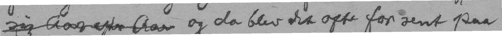sig Aar efter Aar og da blev det ofte for sent paa
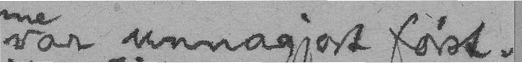var unnagjort først.
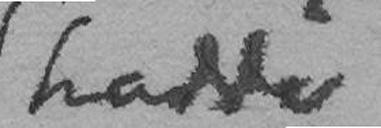hadde
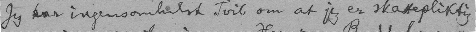Jeg har ingensomhelst Tvil om at jeg er skattepliktig
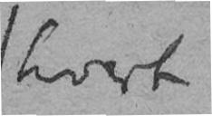hvert
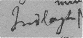Indlagt
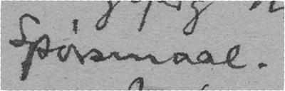Spørsmaal.
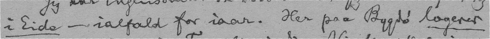i Eide - ialfald for iaar. Her paa Bygdø logerer
